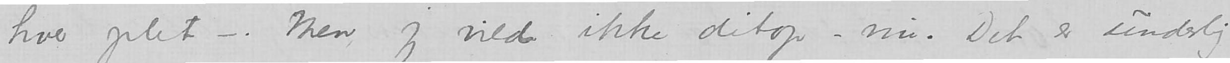hver plet –. Men jeg vilde ikke ditop - nu. Det er underlig
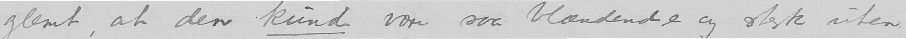glemt, at den kunde være saa blændende og sterk uten
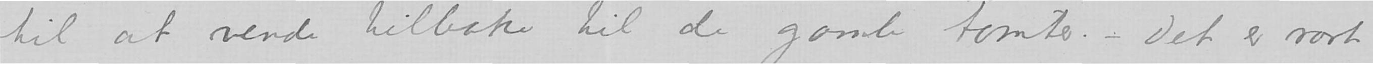til at vende tilbake til de gamle tomter. - Det er rart
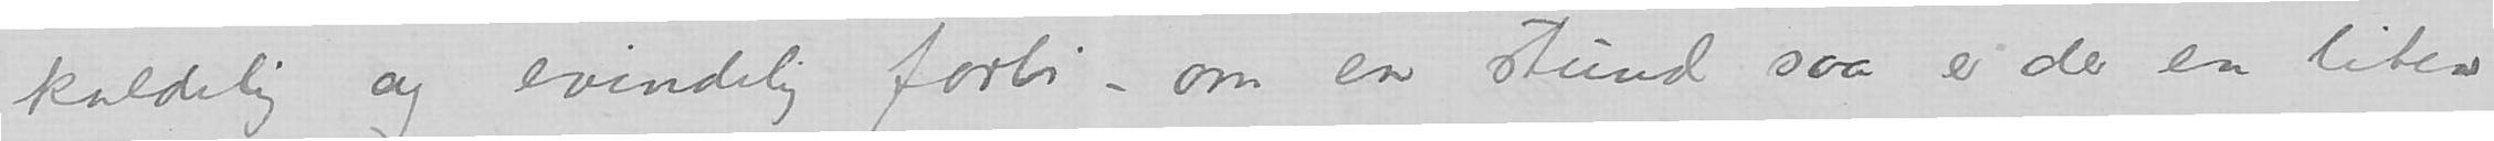kaldelig og evindelig forbi – om en stund saa er der en liten
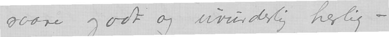saare godt og uvurderlig herlig -.
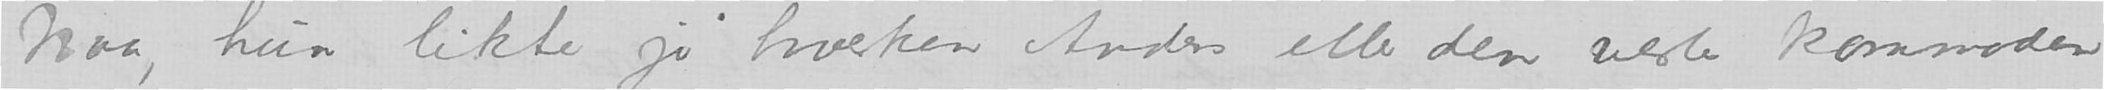Naa, hun likte jo hverken Anders eller den vesle kommoden
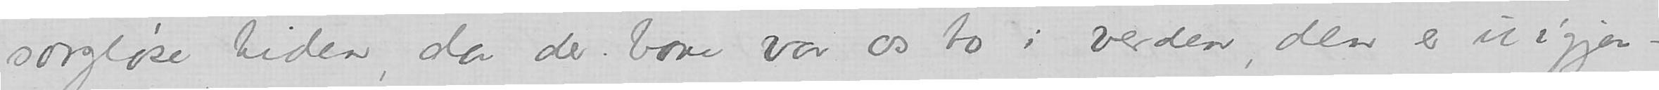sorgløse tiden, da der bare var os to i verden, den er uigjen-
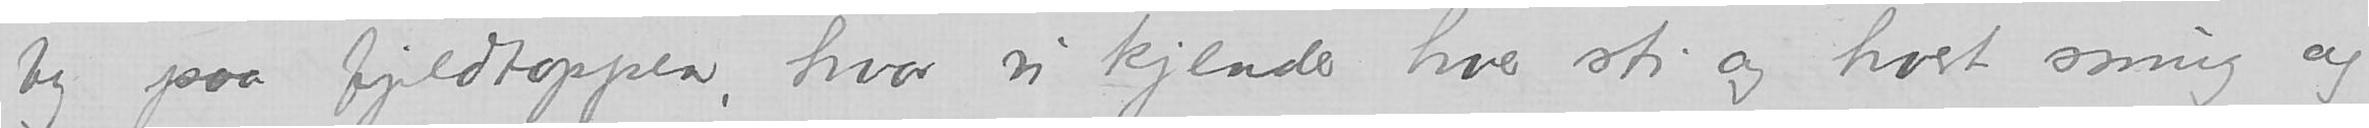by paa fjeldtoppen, hvor vi kjender hver sti og hvert smug og
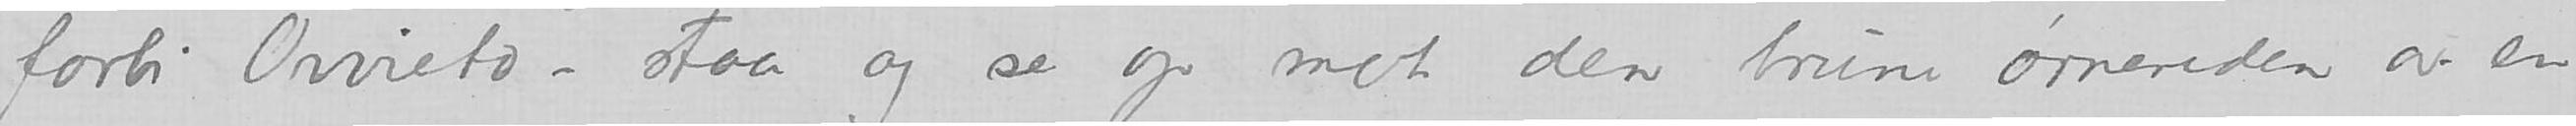forbi Orvieto – staa og se op mot den brune ørnereden av en
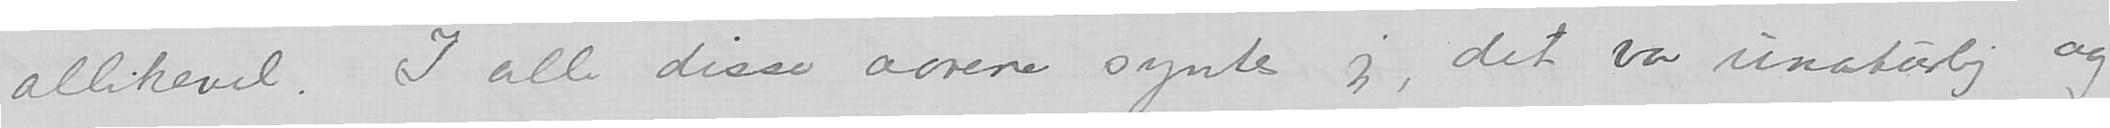allikevel. I alle disse aarene syntes jeg, det var unaturlig. og
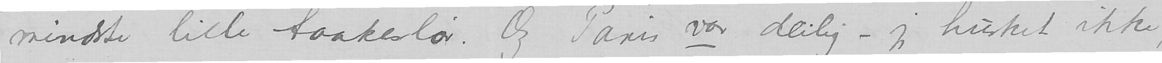mindste lille taakeslør. Og Paris var deilig - jeg husket ikke
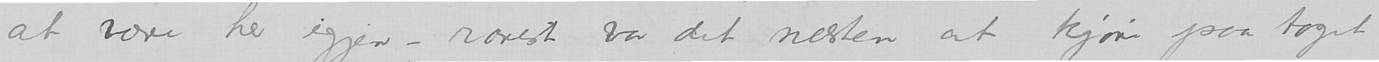at være her igjen - rarest var det næsten at kjøre paa toget
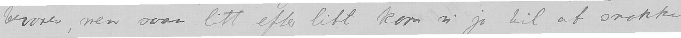bevares, men saan litt efter litt kom vi jo til at snakke
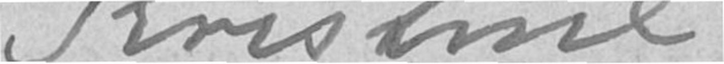Kristine
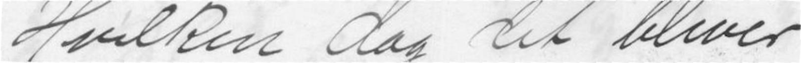Hvilken dag det bliver
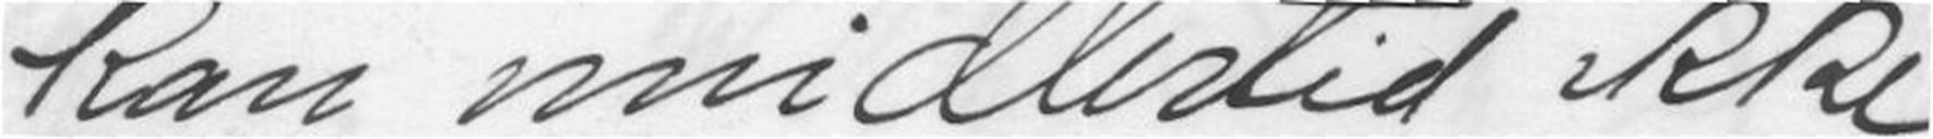kan imidlertid ikke
